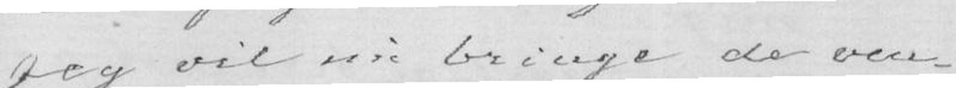Jeg vil nu bringe de ven-
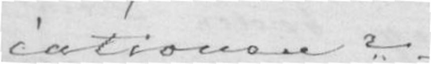cationen?-
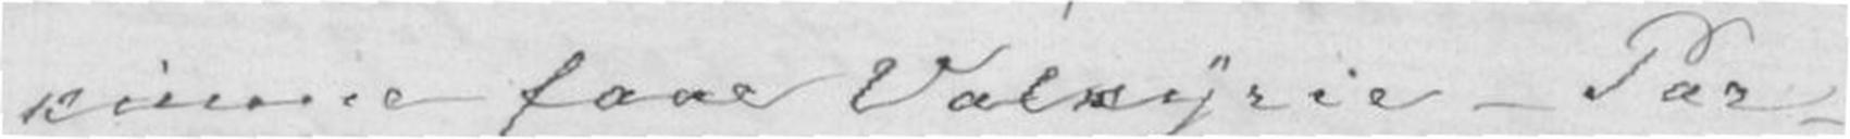kunne faae Valkyrie- Par-
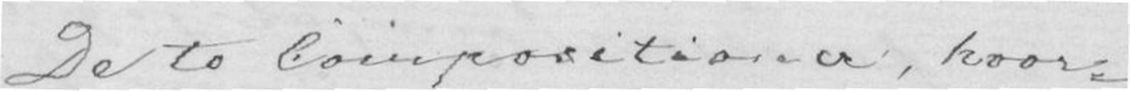De to Compositioner, hvor-
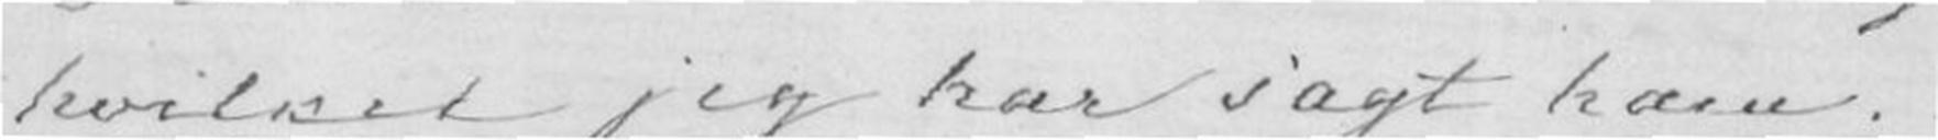hvilket jeg har sagt ham.-
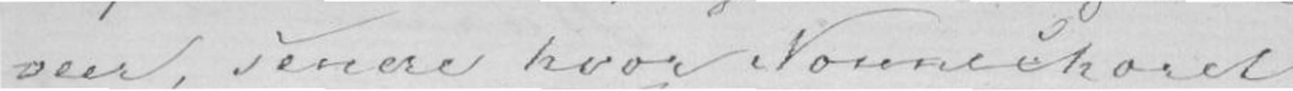-ver, senere hvor Nonnechoret
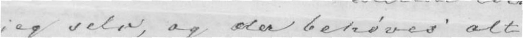jeg selv, og der behøves alt-
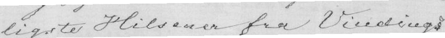ligste Hilsener fra Vindings
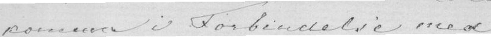kommer i Forbindelse med
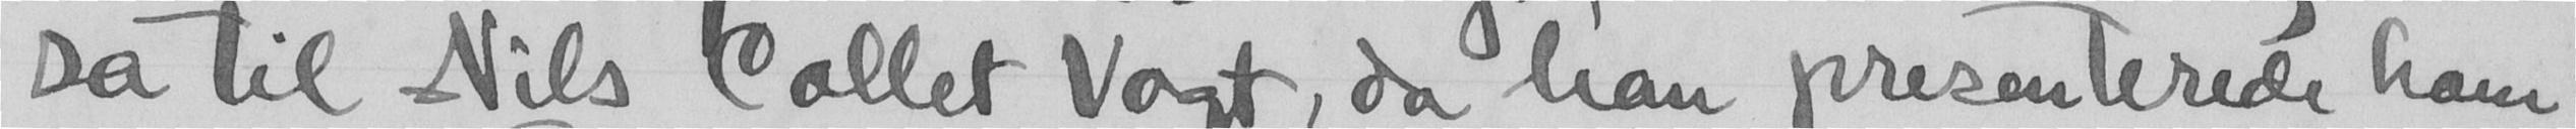sa til Nels Collet Vogt, da han presenterede ham
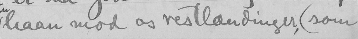haan mod os vestlændinger, (som
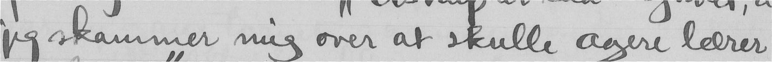jeg skammer mig over at skulle agere lærer
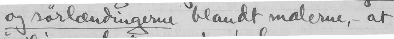og sørlændingerne blandt malerne, - at
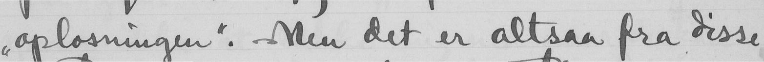"opløsningen". Men det er altsaa fra disse
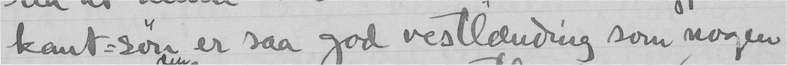kant-søn er saa god vestlænding som nogen
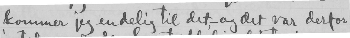kommer jeg endelig til det, - og det var derfor
